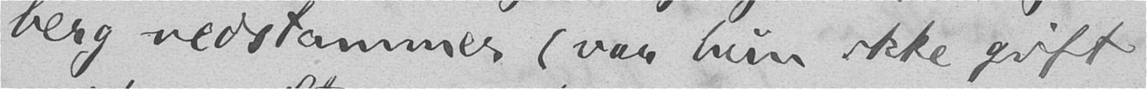berg nedstammer (var hun ikke gift
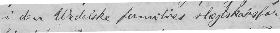i den Wedelske families slægtskabsfor-
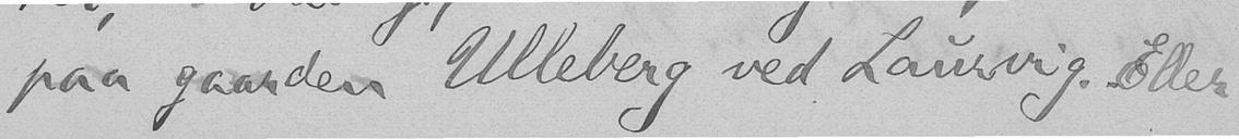paa gaarden Ulleberg ved Laurvig. Eller
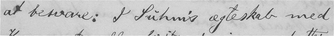at besvare: I Suhm's ægteskab med
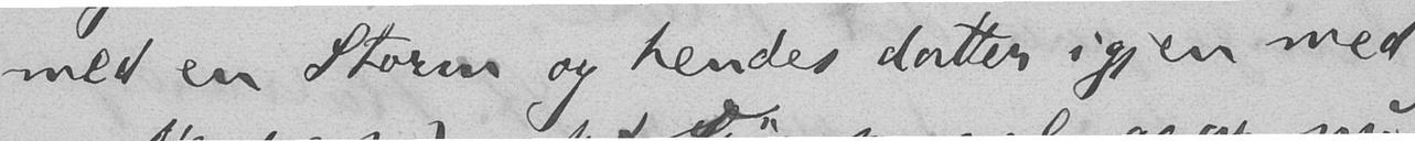med en Storm og hendes datter igjen med
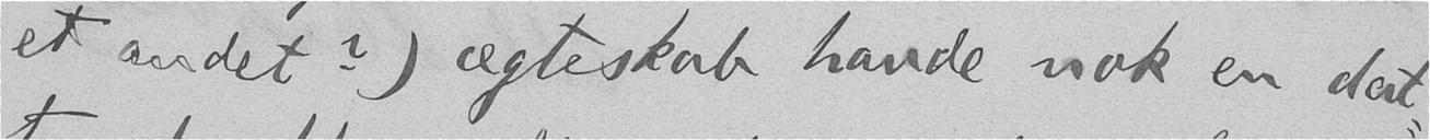et andet?) ægteskab havde nok en dat-
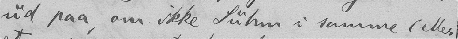ud paa, om ikke Suhm i samme (eller
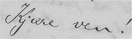Kjære ven!
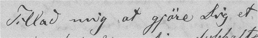Tillad mig at gjøre Dig et
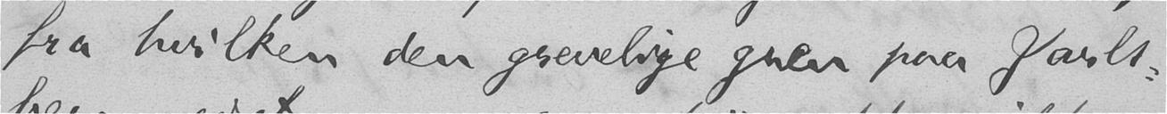fra hvilken den grevelige gren paa Jarls-
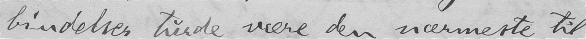bindelser turde være den nærmeste til
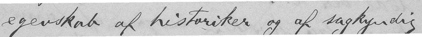egenskab af historiker og af sagkyndig
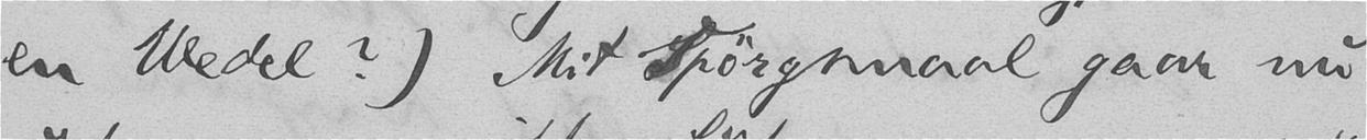en Wedel?) Mit spørgsmaal gaar nu
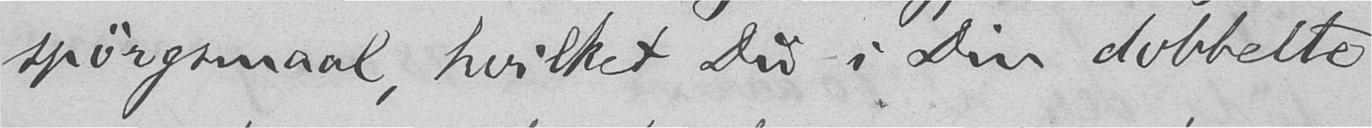spørgsmaal, hvilket Du i Din dobbelte
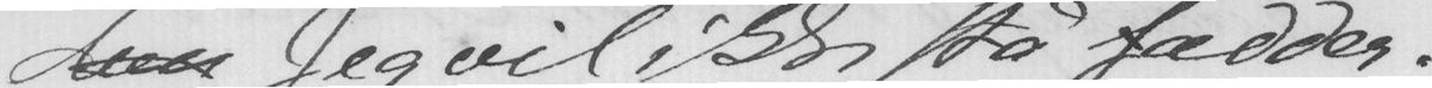Men Jeg vil ikke stå fadder.
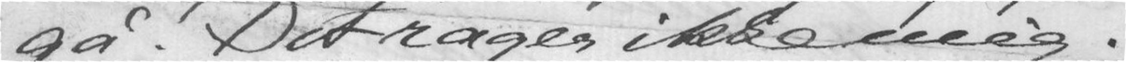lad gå! Det rager ikke mig.
